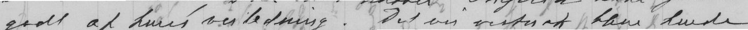godt af hans veiledning. Det vil vistnok blive hende
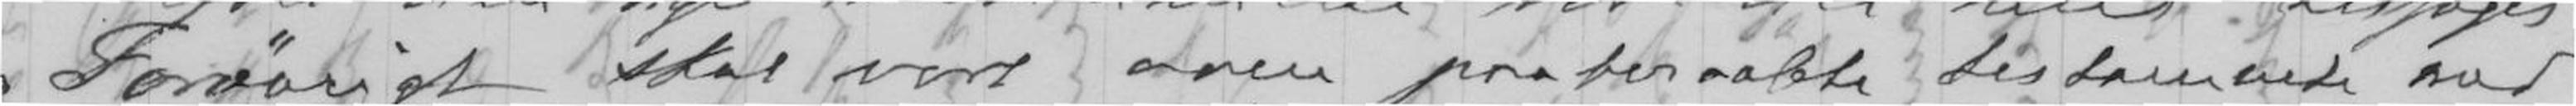Forøvrigt skal vort oven paaberaabte testamente med
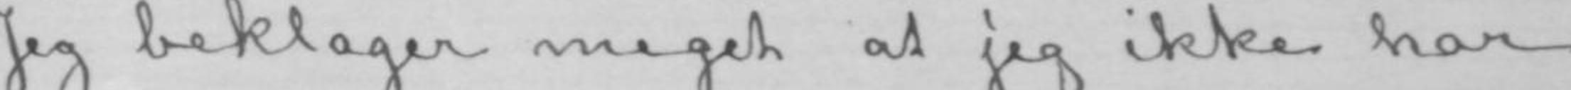Jeg beklager meget at jeg ikke har
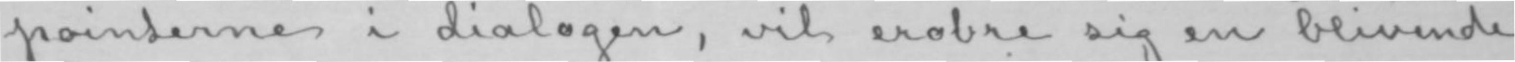pointerne i dialogen, vil erobre sig en blivende
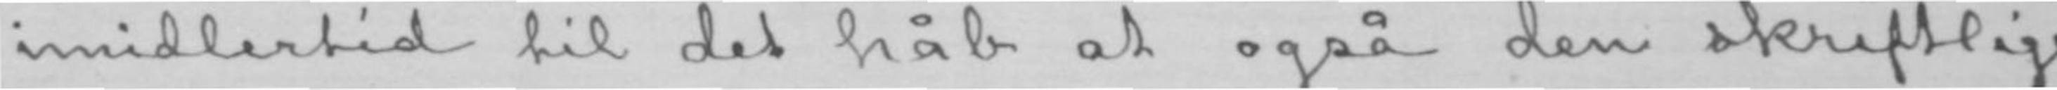imidlertid til det håb at også den skriftlige
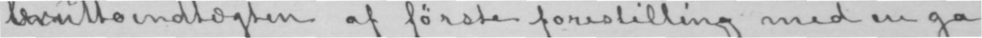inbruttoindtægten af første forestilling med en ga-
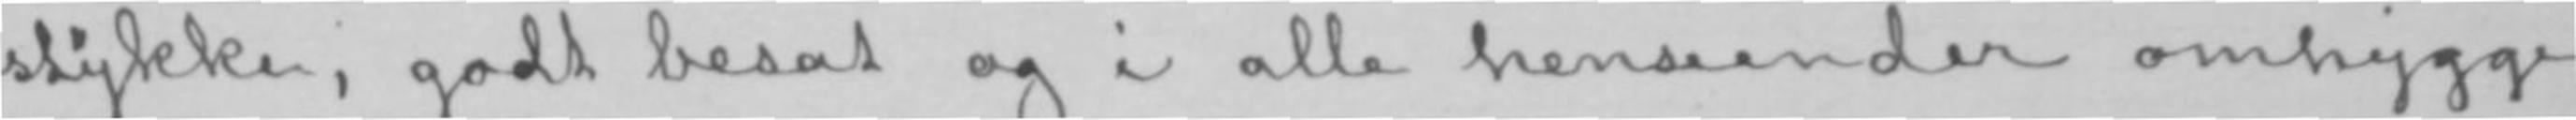stykke, godt besat og i alle henseender omhygge-
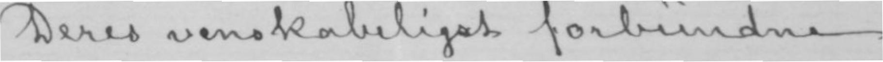Deres venskabeligst forbundne
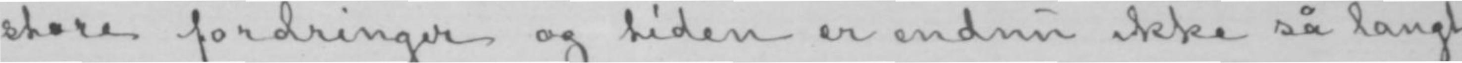store fordringer og tiden er endnu ikke så langt
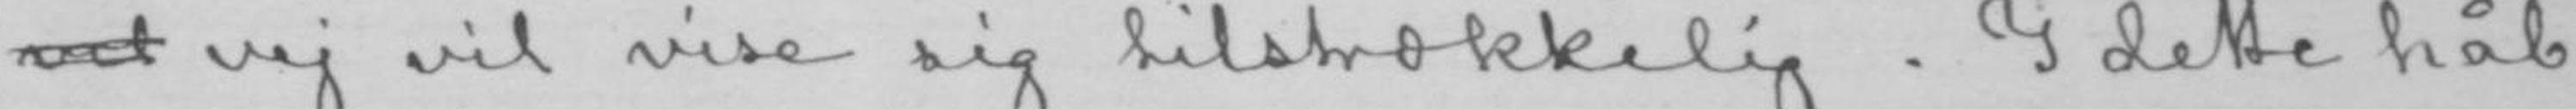vil vej vil vise sig tilstrækkelig. I dette håb
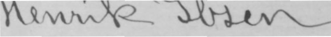Henrik Ibsen.
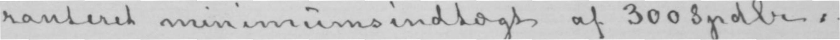ranteret minimumsindtægt af 300 Spdlr:-
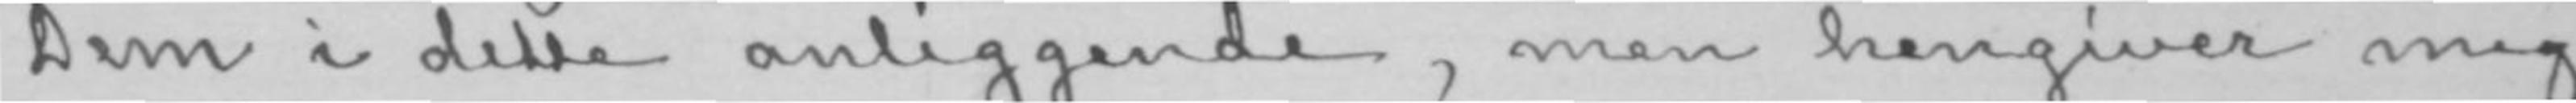Dem i dette anliggende, men hengiver mig
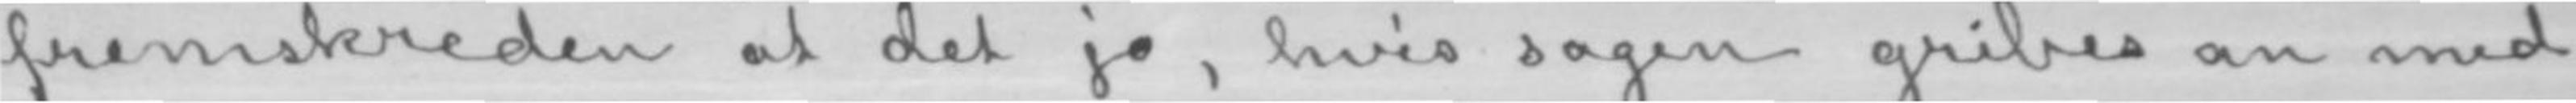fremskreden at det jo, hvis sagen gribes an med
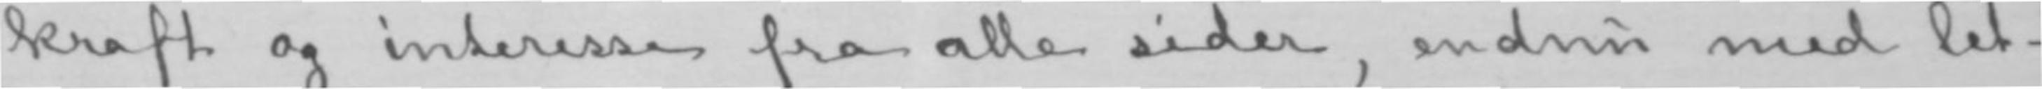kraft og interesse fra alle sider, endnu med let-
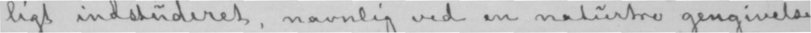ligt indstuderet, navnlig ved en naturtro gengivelse
